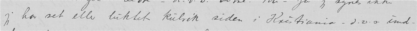jeg har set eller luktet kulrøk siden i Kristiania – d.v.s. undtagen ind
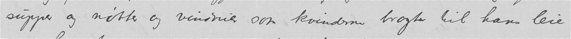supper og nøtter og vindruer som kvinderne bragte til hans leie
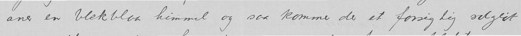aner en blekkblaa himmel og saa kommer der et forsigtig solgløt
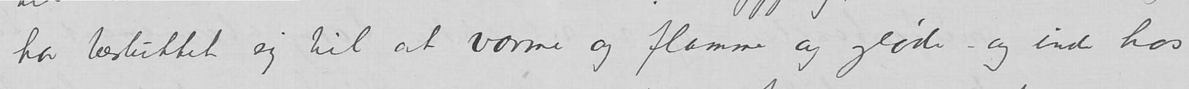har besluttet sig til at varme og flamme og gløde – og inde hos
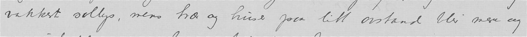vakkert sollys, mens trær og huse paa litt avstand blir mere og
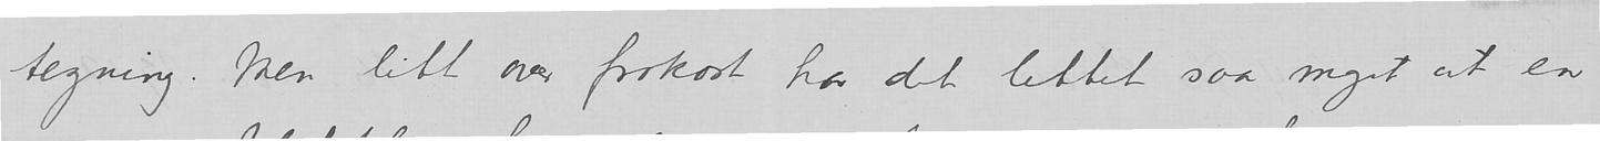tegning. Men litt over frokost har det lettet saa meget at en
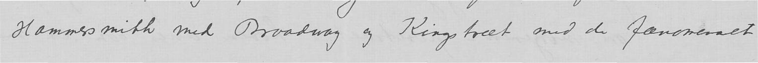Hammersmith med Broadway og Kingstreet med de fænomenalt
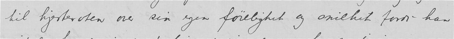til hjerteroten over sin egen føielighet og snilhet fordi han
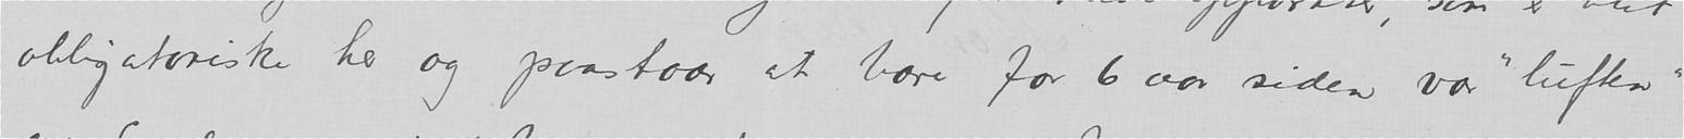obligatorisk her og paastaar at bare for 6 aar siden var «luften»
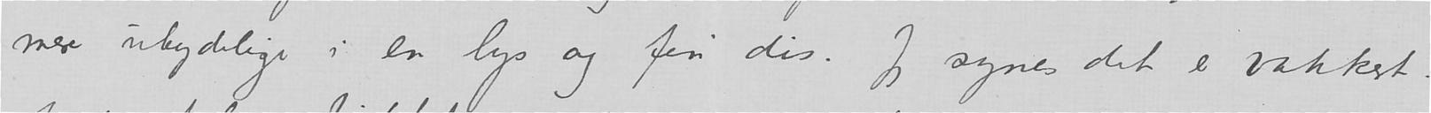mere utydelige i en lys og fin dis. Jeg synes det er vakkert.
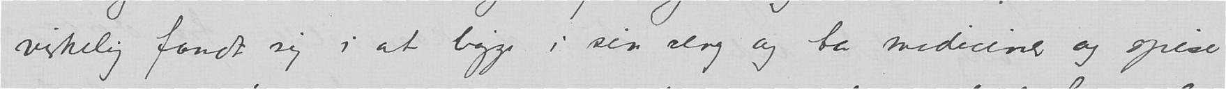virkelig fandt sig i at ligge i sin seng og ta mediciner og spise
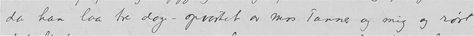da han laa tre dager – opvartet av mrs Tanner og mig og rørt
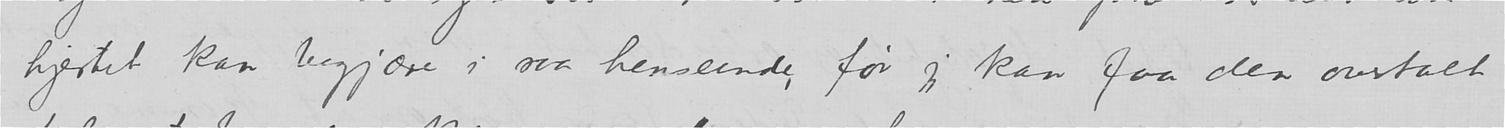hjertet kan begjære i saa henseende, før jeg kan faa den overtalt
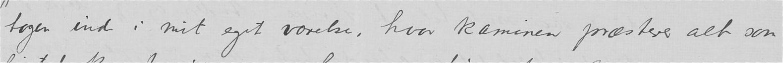i mit eget værelse, hvor kaminen præsterer alt som
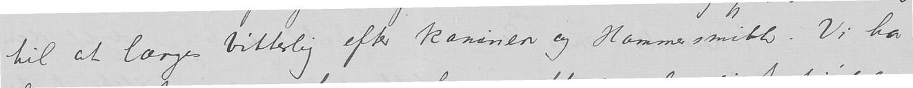til at længes bitterlig efter kaminen og Hammersmith. Vi har
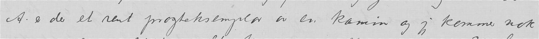A. er der et rent pragteksemplar av en kamin og jeg kommer nok
